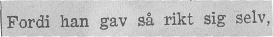Fordi han gav så rikt sig selv,
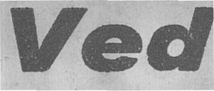Ved
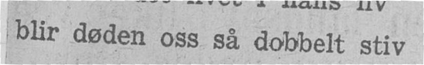blir døden oss så dobbelt stiv
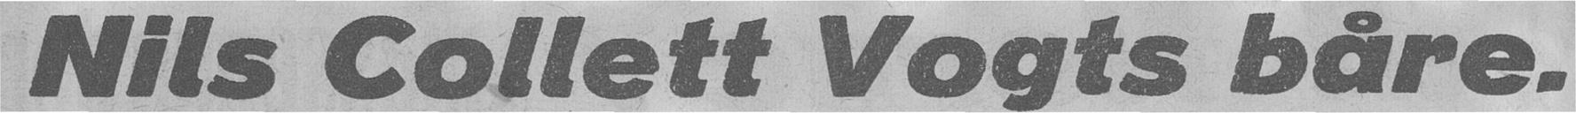Nils Collett Vogts båre.
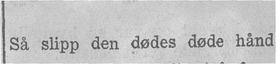Så slipp den dødes døde hånd
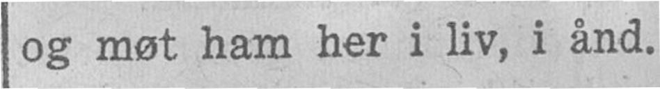og møt ham her i liv, i ånd.
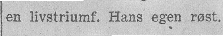en livstriumf. Hans egen røst.
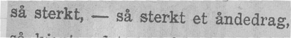så sterkt, — så sterkt et åndedrag,
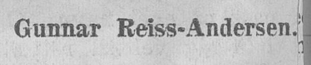Gunnar Reiss-Andersen.
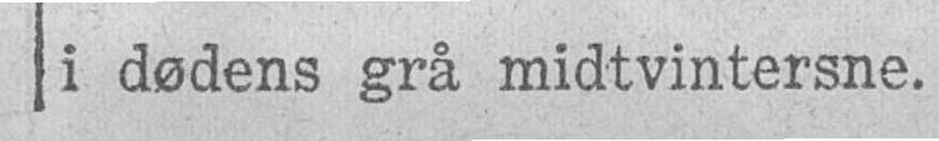i dødens grå midtvintersne.
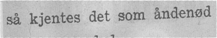så kjentes det som åndenød
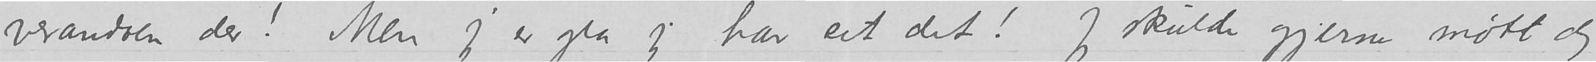verandaen der! Men jeg er gla jeg har set det! Jeg skulde gjerne møtt dig
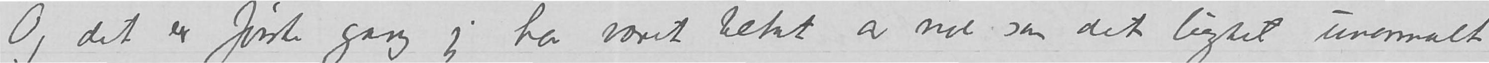Og det er første gang jeg har været betat av noe som det lugtet unormalt
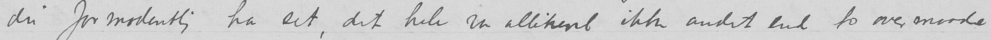formodentlig ha set, det hele var allikevel ikke andet end to overmaade
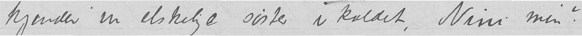kjender vor elskelige søster i kaldet, Nini min?
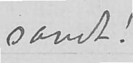sandt!
